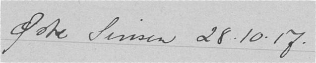Østre Sinsen 28.10.17.
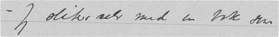- Jeg sliter selv med en bok som
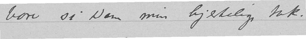bare si Dem min hjertelige tak.
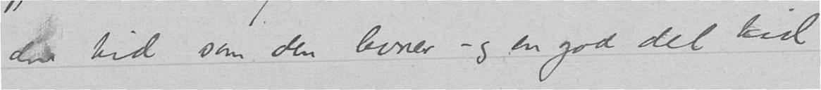den tid som den levner – og en god del tid
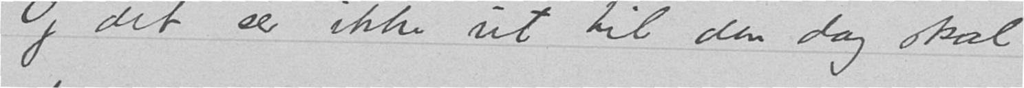Og det ser ikke ut til den dag skal
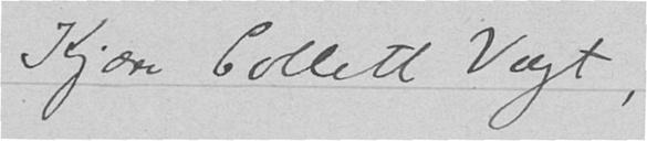Kjære Collet Vogt,
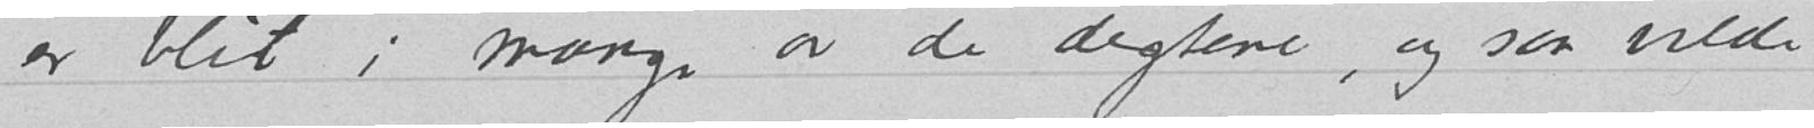er blit i mange av de digtene, og saa vilde
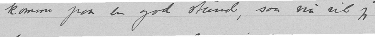komme paa en god stund, saa nu vil jeg
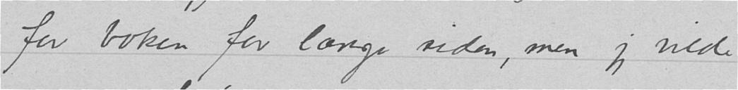for boken for længe siden, men jeg vilde
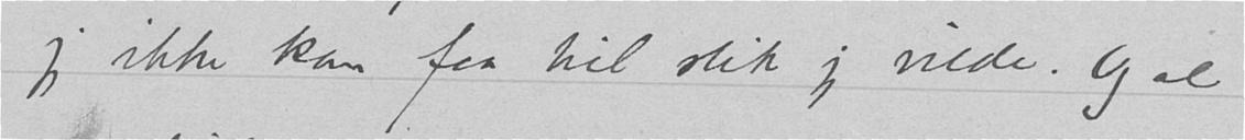jeg ikke kan faa til slik jeg vilde. Og al
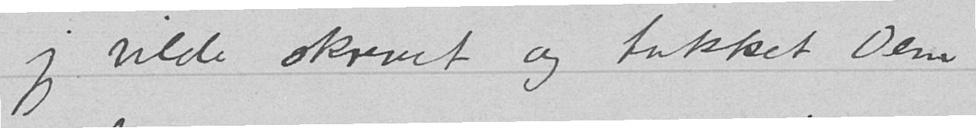jeg vilde skrevet og takket Dem
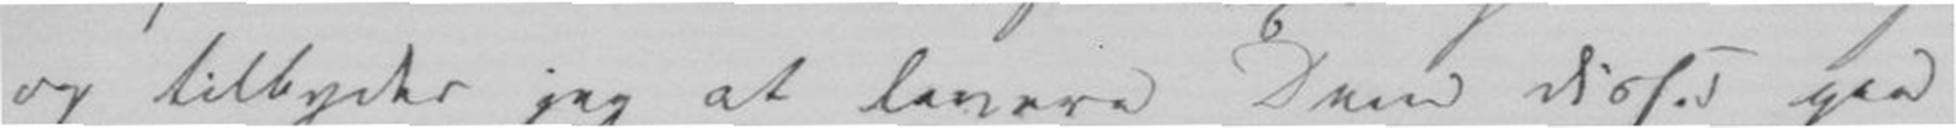og tilbyder jeg at levere Dem disse paa
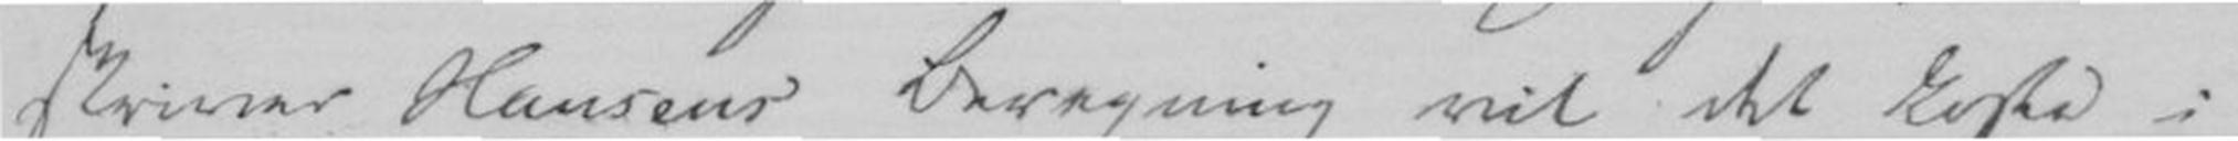skriver Hansen Beregning vil det koste i
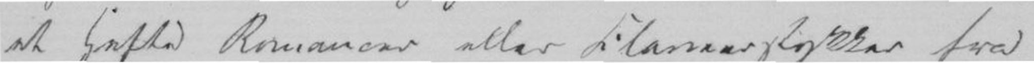et hefte Romancer eller Claverstykker fra
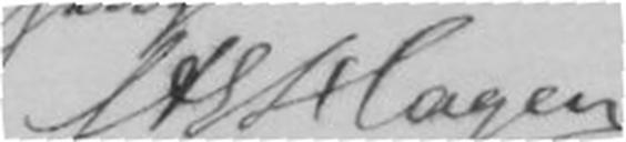S A E Hagen
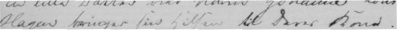Hagen bringer sin Hilsen til Deres Kone.
